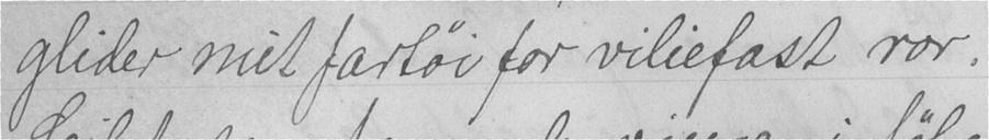glider mit fartøi for viliefast ror.
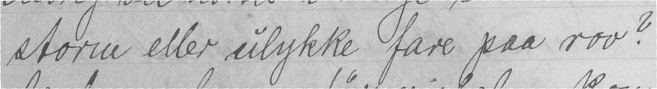storm eller ulykke fare paa rov?
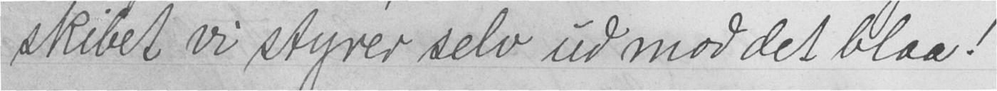skibet vi styrer selv ud mod det blaa!
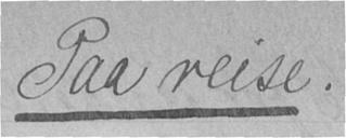Paa reise.
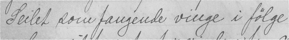Seilet som fangende vinge i følge
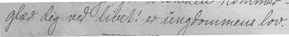glæd dig ved livet! er ungdommens lov.
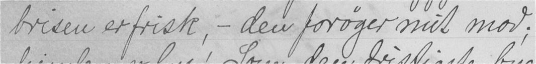brisen er frisk, - den forøger mit mod;
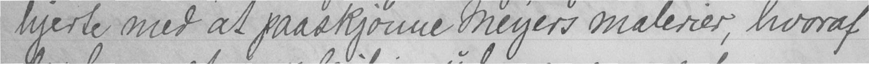hjerte med at paaskjønne Meyers malerier, hvoraf
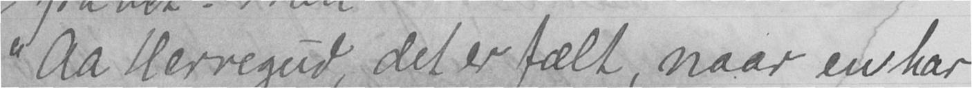"Aa Herregud, det er fælt, naar en har
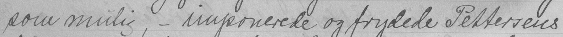som mulig, - imponerede og frydede Pettersens
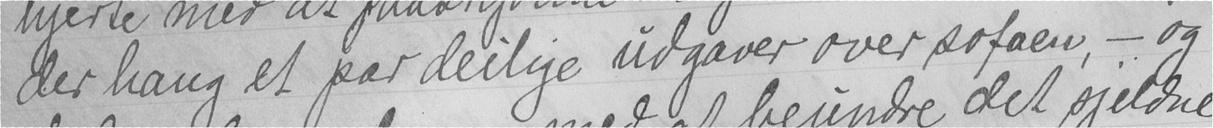der hang et par deilige udgaver over sofaen, - og
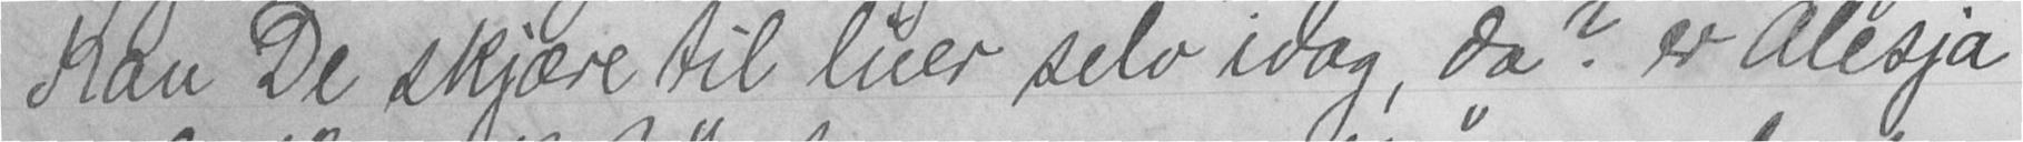Kan De skjære til luer selv idag, da? er Alesja
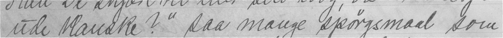ude kanske?" saa mange spørgsmaal som
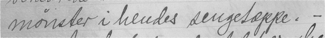mønster i hendes sengetæppe. -
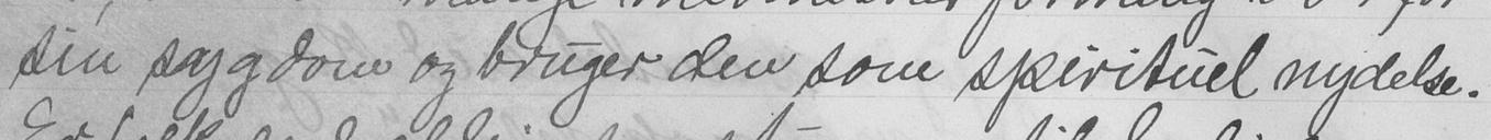sin sygdom og bruger den som spirituel nydelse.
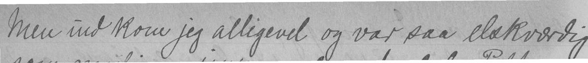Men ind kom jeg alligevel og var saa elskværdig
